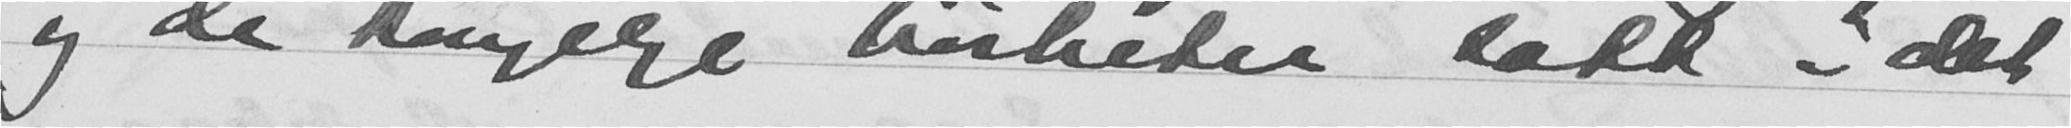og de kongelige høiheter satt - det
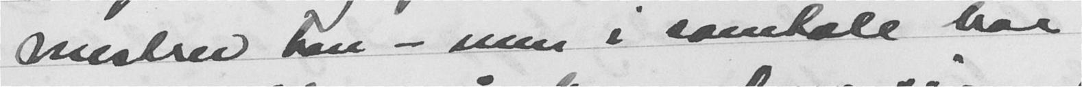mestrer han - men i samtale har
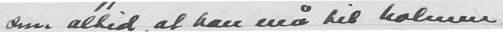som altid at han må til holmen
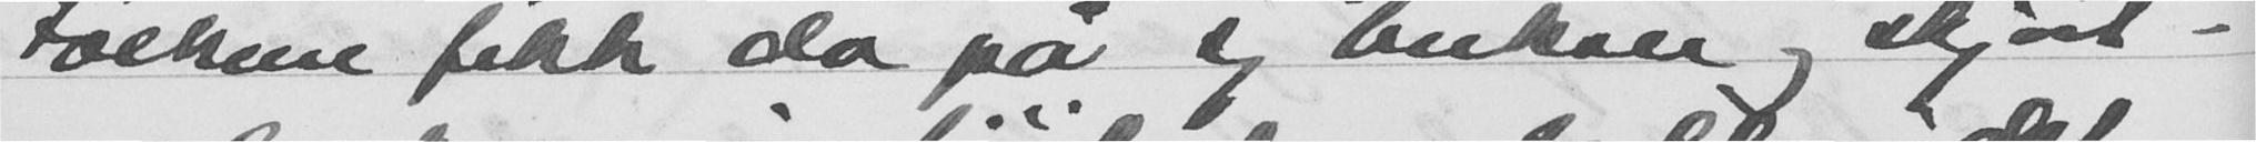Folkene fikk da på sig bukser og skjørt -
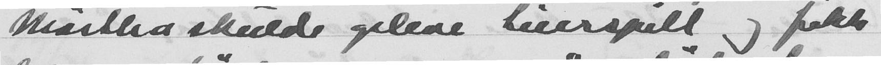Märths skulde opleve livsspill og fikk
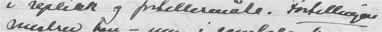i replikk og fortellermåte. Fortellingen
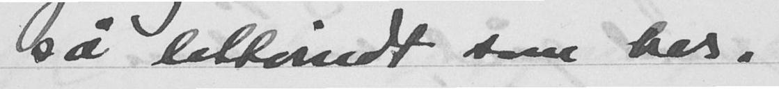så lettvindt som her.
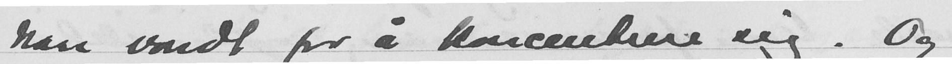han vondt for i koncentrere sig. Og
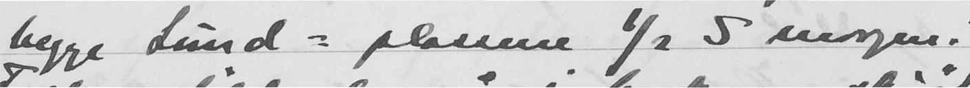begge Lund- plassene 1/2 5 morgen.
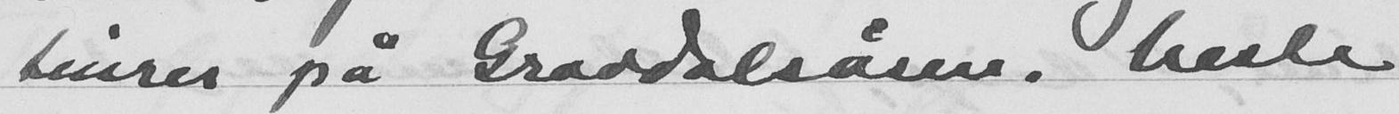linser pa Gravdalsåsen. Neste
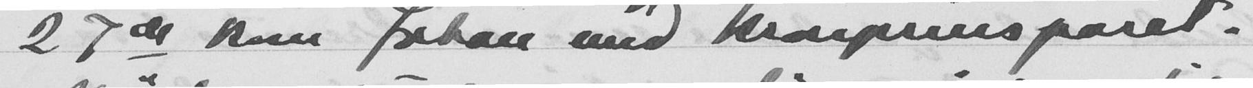27de kom Johan med kronprinsparet.
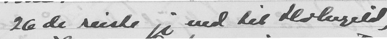26de reiste jeg ned til Holmgild,
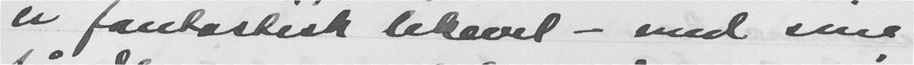er fantastisk likevel - med sine
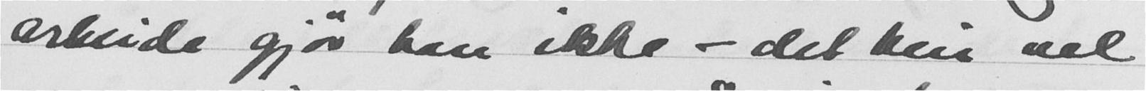arbeide gjør han ikke - det blir vel
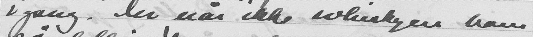i gang. Lei når ikke whiskyen kom
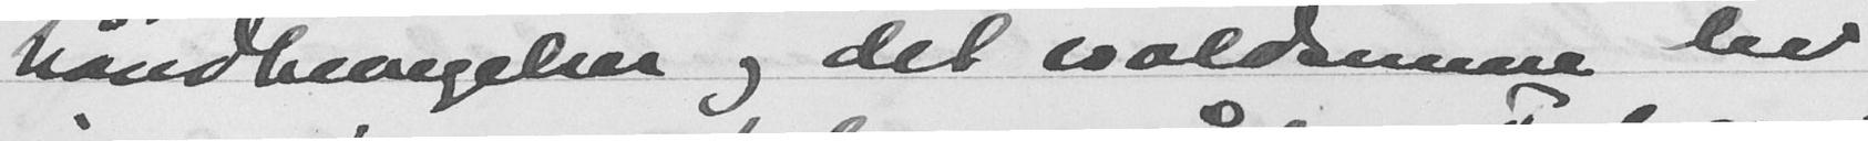håndbevegelser og det voldsomme liv
